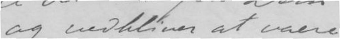og vedbliver at vaere
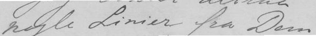nogle Linier fra Dem
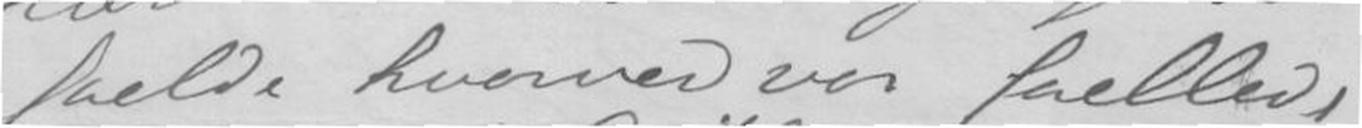faelde hvormed vor faelleds
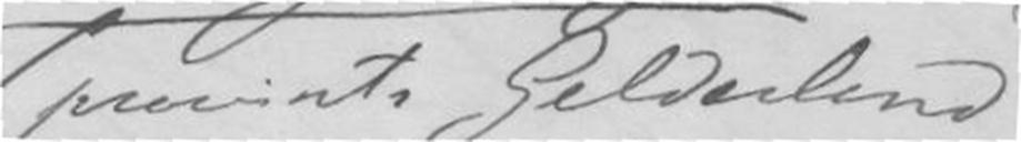(provints Gelderlend )
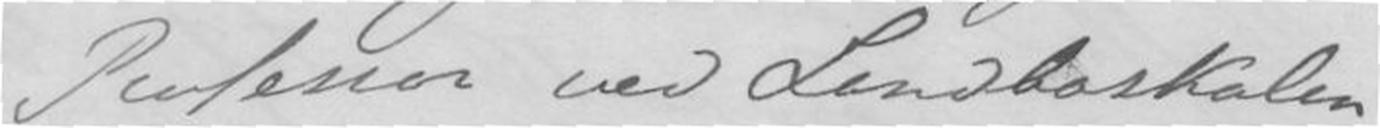Professor ved Landboskolen
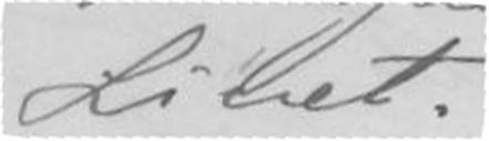Livet
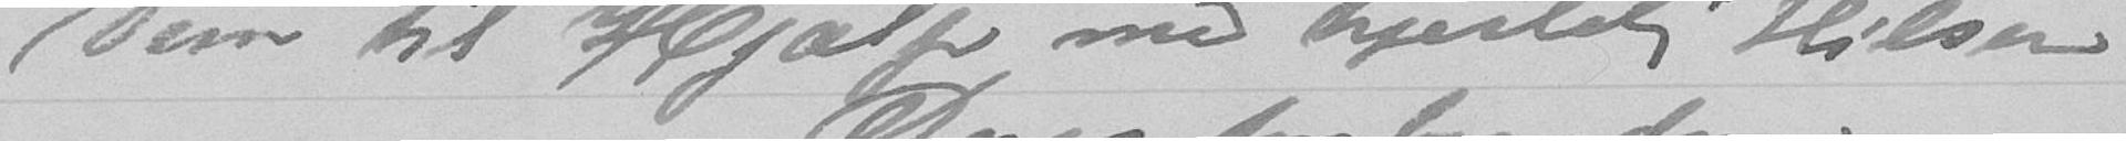Dem til Hjælp med hjertelig Hilsen
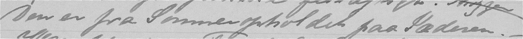Den er fra Sommeropholdet paa Sæderen. -
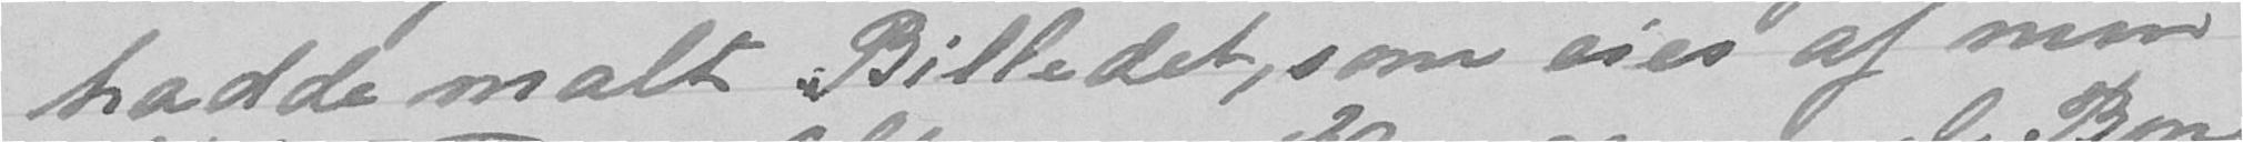hadde malt Billedet, som eies af min
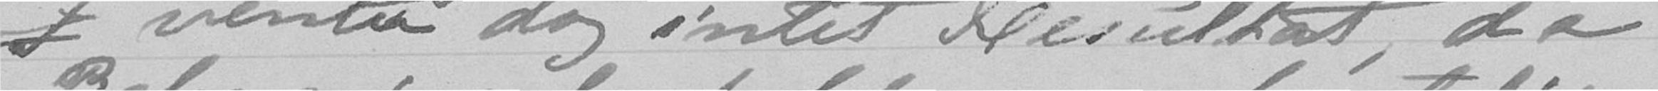t venter dog intet Resultat, da
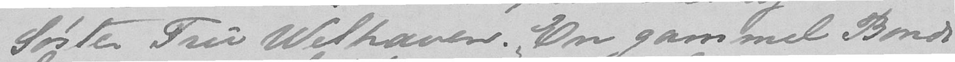Søster Fru Welhaven. En gammel Bonde
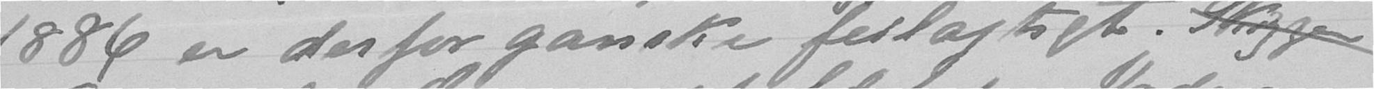1886 er derfor ganske feilagtigt. Skizzen
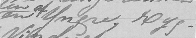en yngre, Ryg-
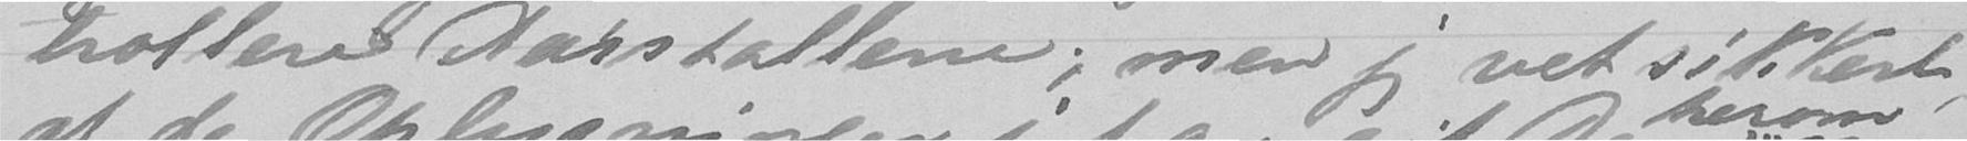trollere Aarstallene; men jeg vet sikkert
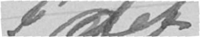I det
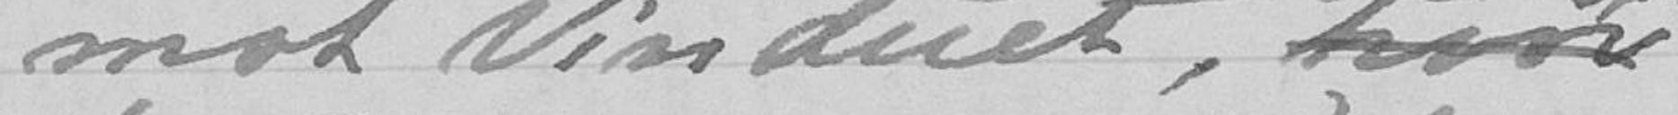mot Vinduet, hvor
